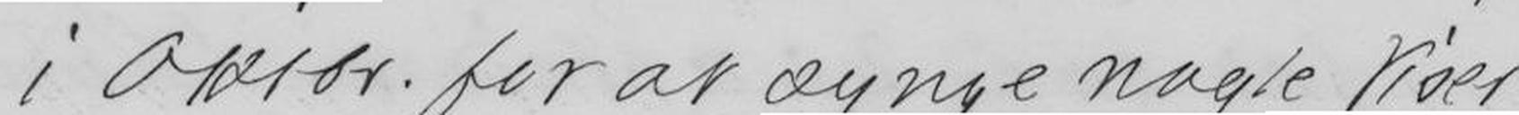i Oktobr. for at synge nogle viser
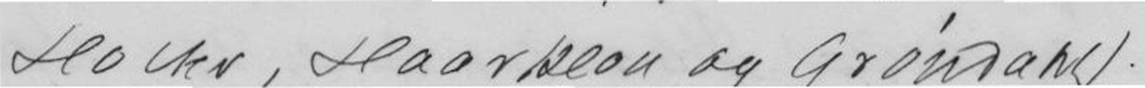Holter, Haarklon og Grøndahl,
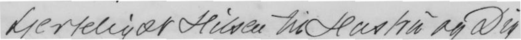hjerteligst Hilsen til Hustru og Dig
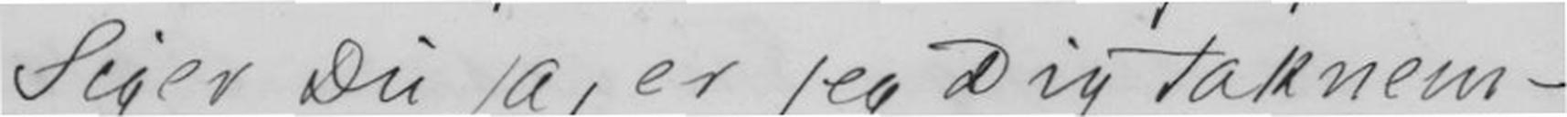Siger Du ja, er jeg Dig taknem-
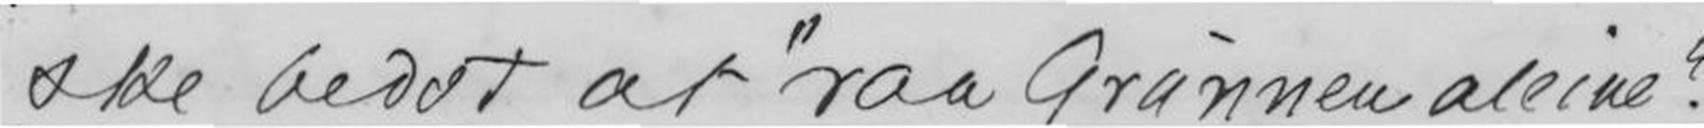ske bedst at " saa Grunnen aleine".
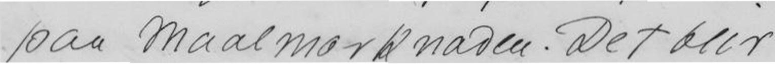paa maalmorknaden. Det blir
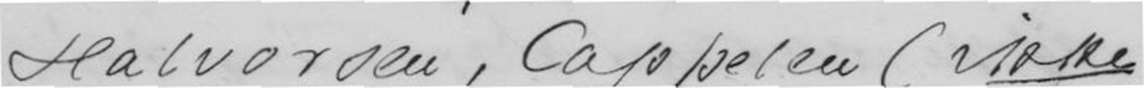Halvorsen, Cappelen (ikke
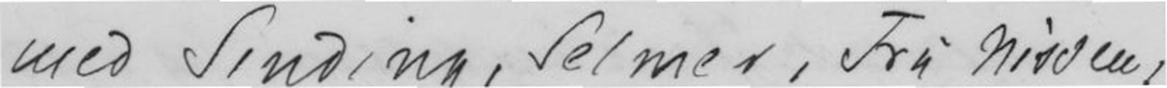med Sinding, Selmer, Fru Nissen,
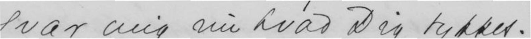Svar mig nu hvad Dig tykkes.
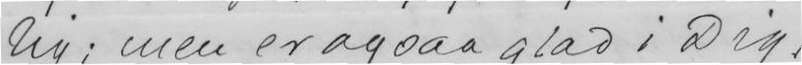lig; men er ogsaa glad i Dig,
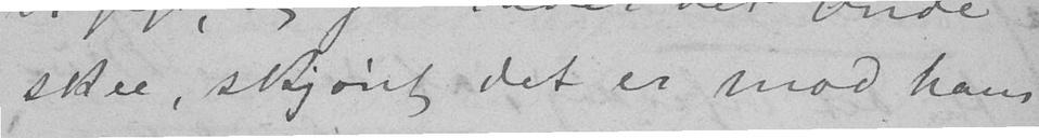skee, skjønt det er mod hans
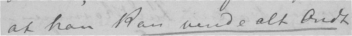at han kan vende alt Ondt
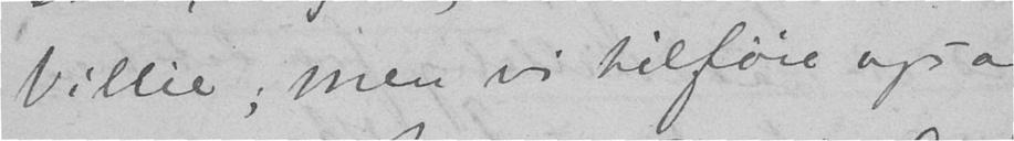Villie, men vi tilføie ogsaa,
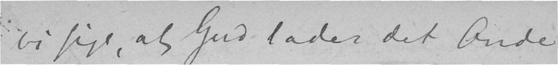vi sige, at Gud lader det Onde
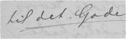til det Gode
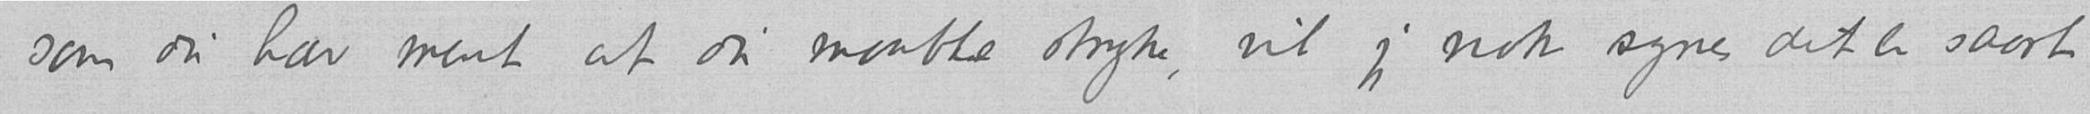som du har ment at du maatte stryke, vil jeg nok synes det er saart
